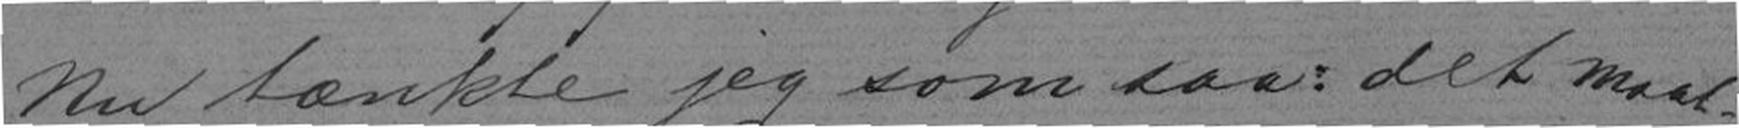Nu tænkte jeg som saa: det maat-
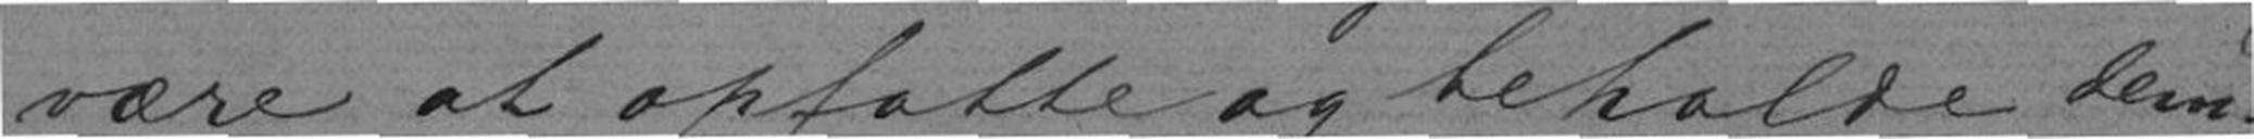være at opfatte og beholde dem.
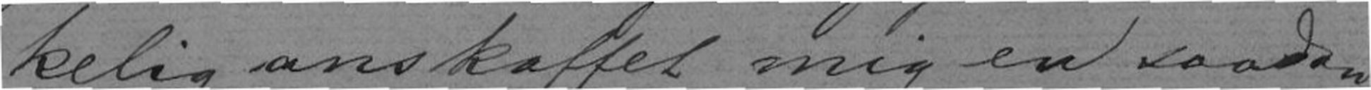kelig anskaffet mig en saadan,
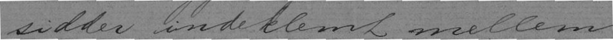sidder indeklemt mellem
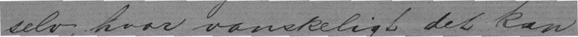selv, hvor vanskeligt det kan
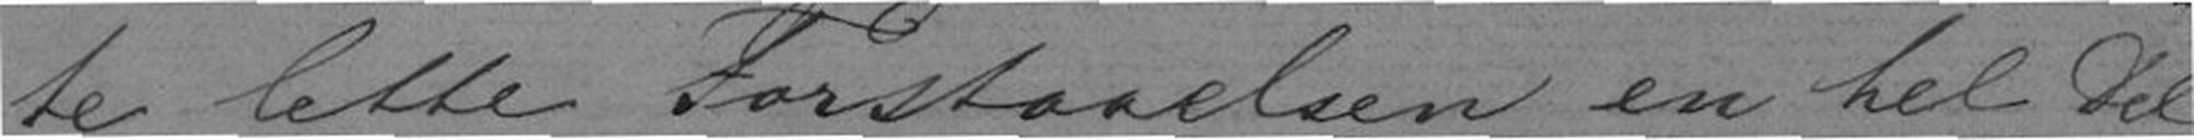te lette Forstaaelsen en hel Del,
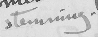stemning.
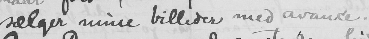sælger mine billeder med avance.
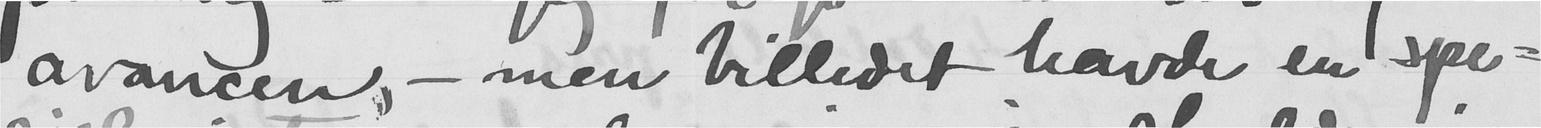avancen, - men billedet havde en spe-
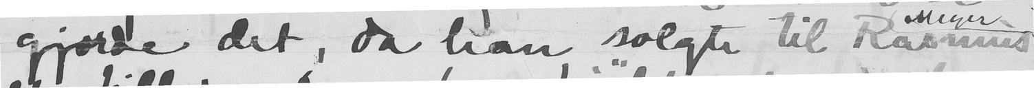gjorde det, da han solgte til Rasmus
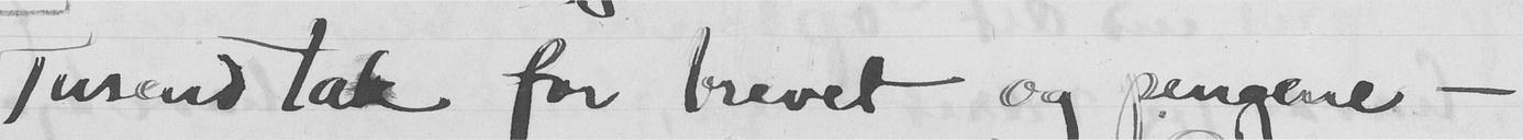Tusend tak for brevet og pengene -
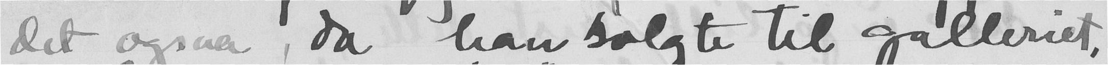det ogsaa, da han solgte til galleriet,
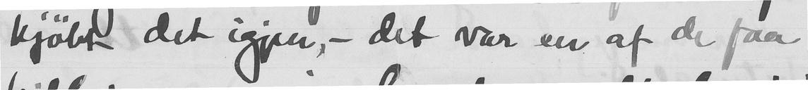kjøbt det igjen, - det var en af de faa
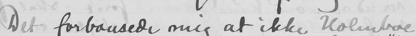Det forbausede mig at ikke Holmboe
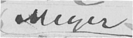Meyer
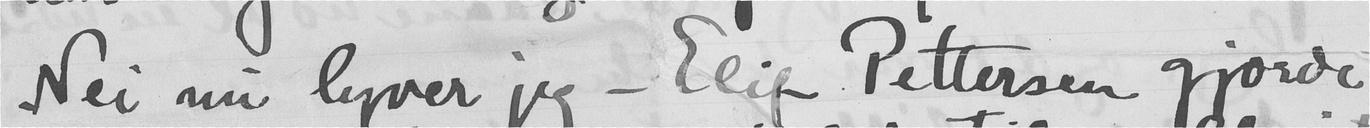Nei nu lyver jeg - Elif Pettersen gjorde
